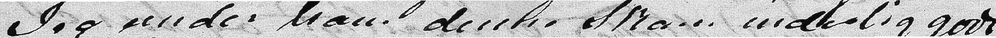Jeg under ham denne Skam inderlig godt,
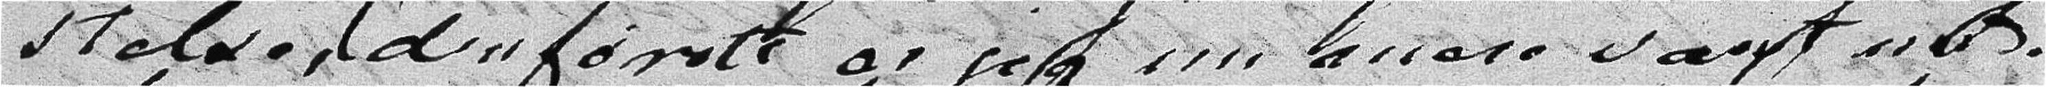stese, den første er jeg nu mere vant med.
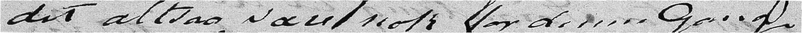det sltsaa være nok for denne Gang.
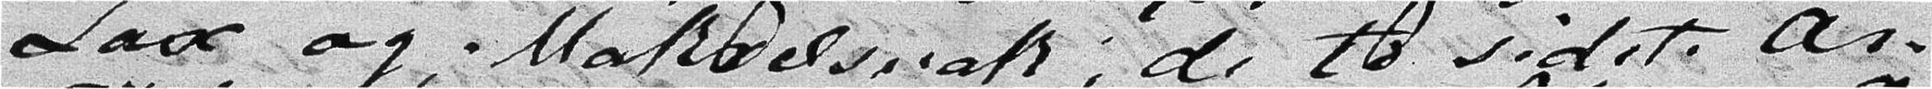Lax og Makrelsnak; de to sidste Ar-
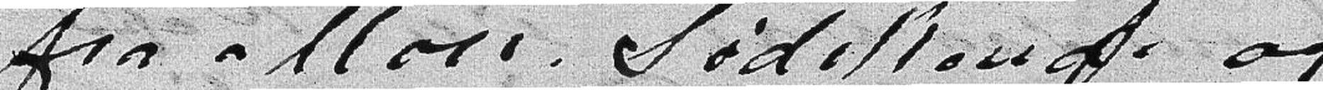fra Moer, Sødsken og
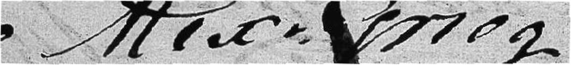Alexr. Grieg
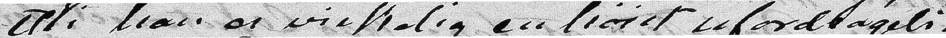thi ham er virkelig en høist ufordragelig
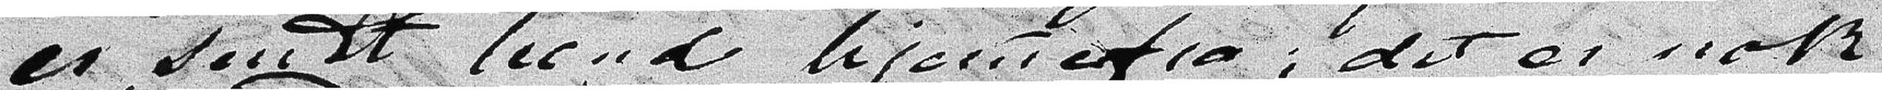er sendt hende hje'mefra; det er nok
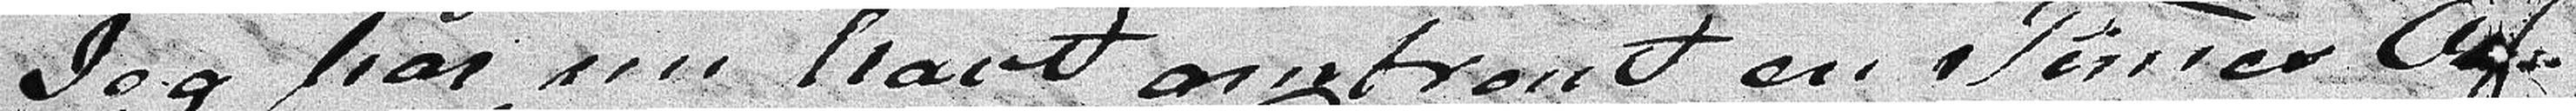Jeg har nu havt omtrent en Times Af-
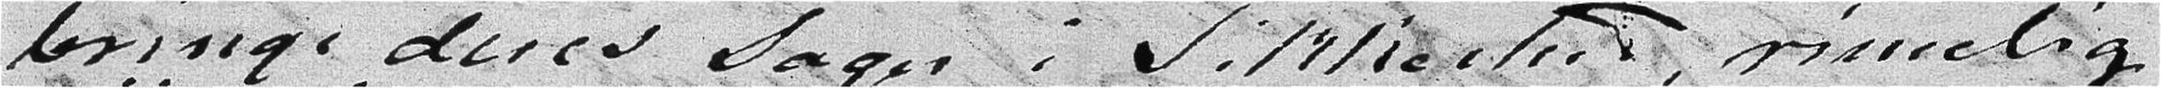bringe deres Sager i Sikkerhed rimelig-
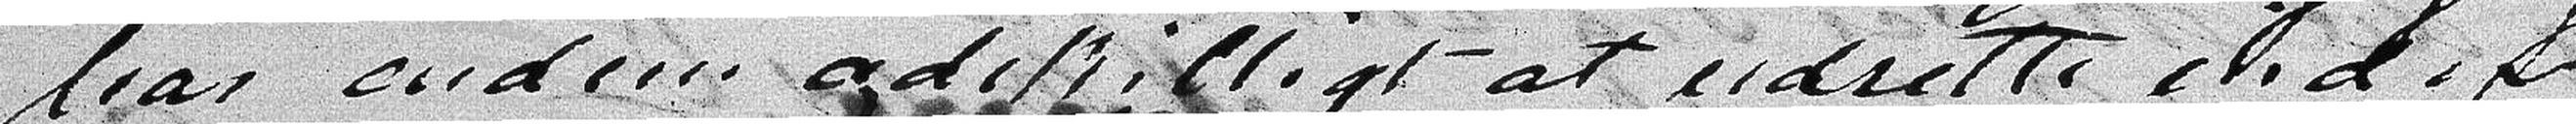har endnu adskilligt at udrette inden
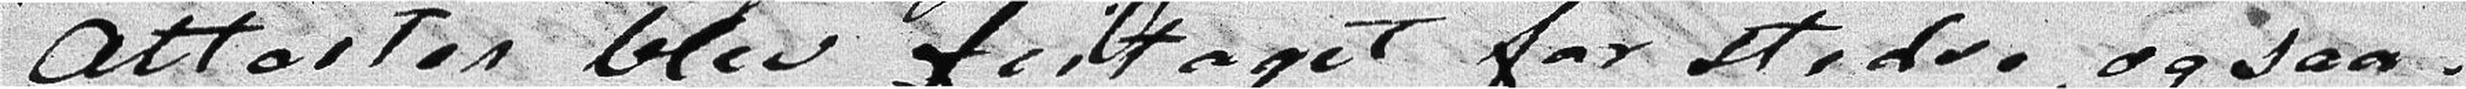Attester blev fritaget for stedse, og saa-
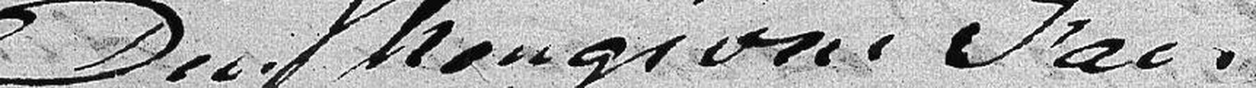Din hengivne Faer
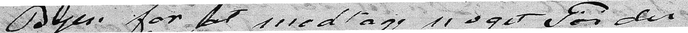Byen for at medtage noget Tøi der
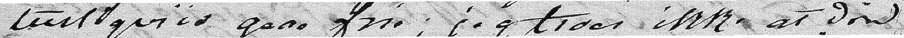turligviis gaae fri; jeg troer ikke at Din
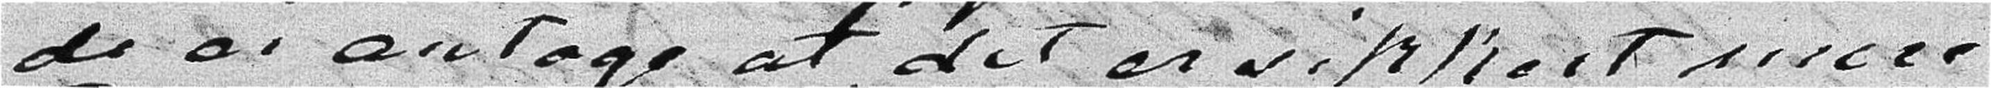de ei antage at det er sikkert mere i
